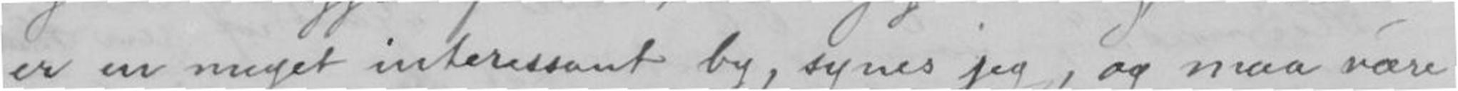er nu meget interessant by, synes jeg, og maa være
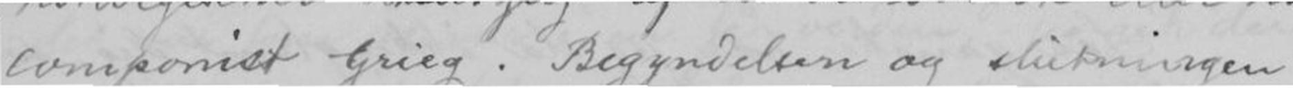componist Grieg. Begyndelsen og slutningen
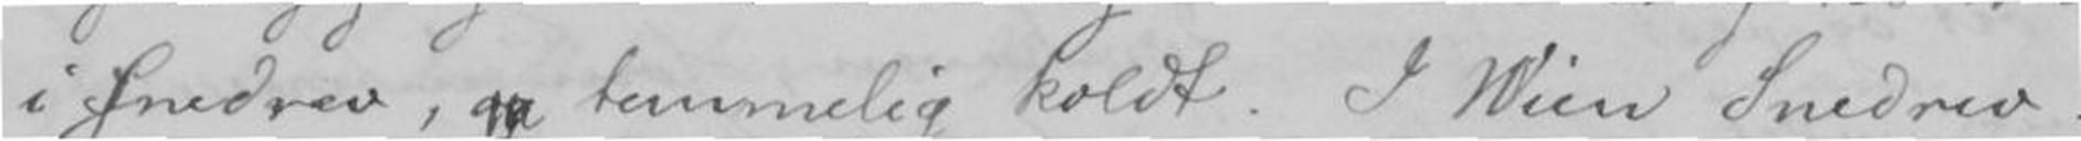i Snedrev, og temmelig koldt. I Wien Snedrev.
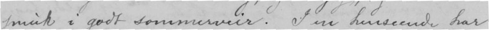smuk i godt sommerveir. I en hensende har
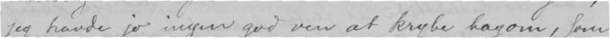jeg havde jo ingen god ven at krybe bagom, som
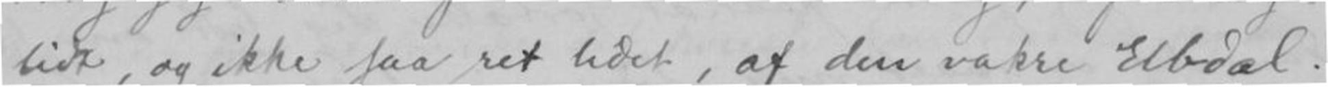lidt, og ikke saa ret lidet, af den vakre Elbdal.
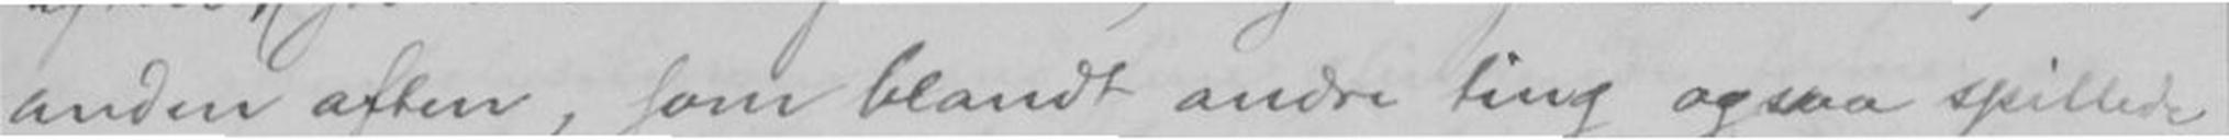anden aften, som blandt andre ting ogsaa spillede
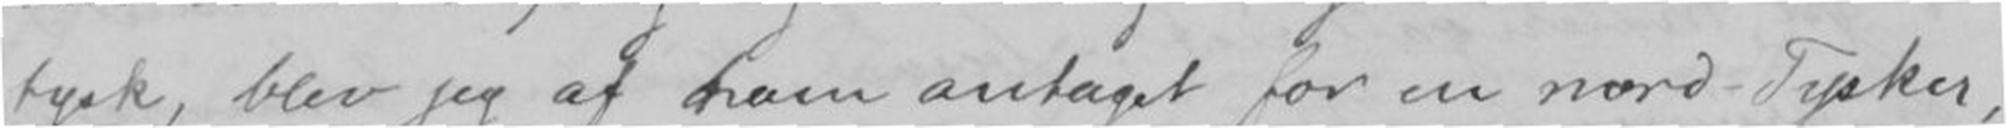tysk, blev jeg af ham antaget for en nord-Tysker,
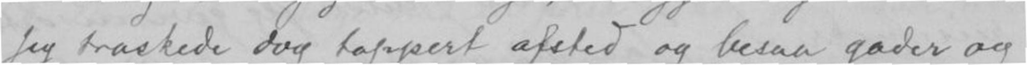Jeg traskede dog tappert afsted og besaa gader og
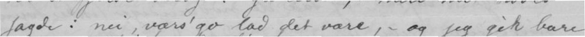sagde: nei, værs'go lad det være,- og jeg gik bare
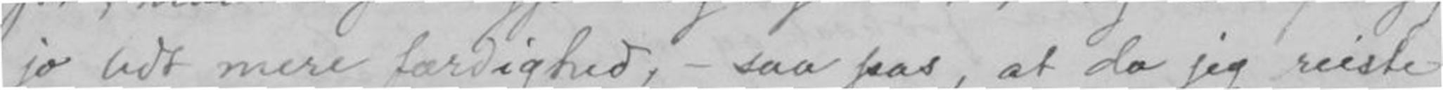jo lidt mere færdighed, - saa pas, at da jeg reiste
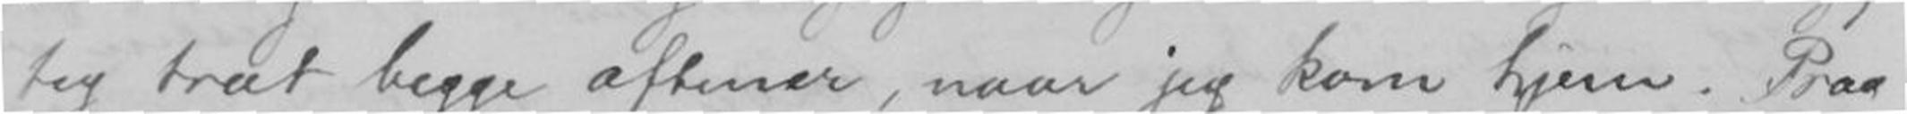tig træt begge aftener, naar jeg kom hjem. Prag
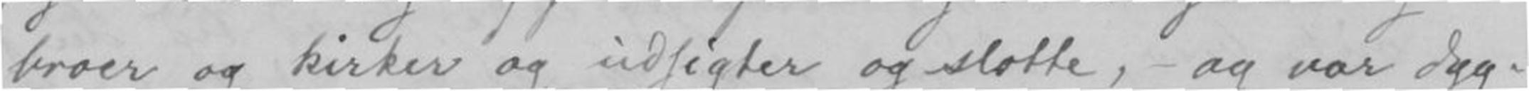broer og kirker og udsigter og slotte, - og var dyg-
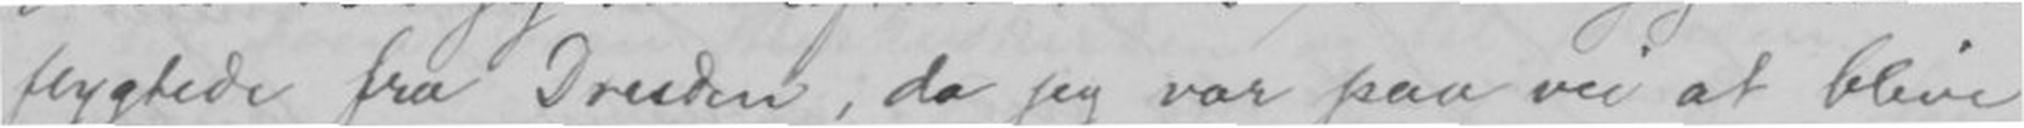flygtede fra Dresden, da jeg var paa vei at blive
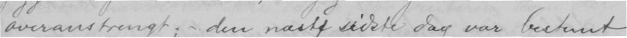overanstrengt; - den nest sidste dag var bestemt
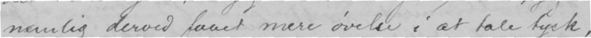næmlig derved faaet mere øvelse i at tale tysk,
